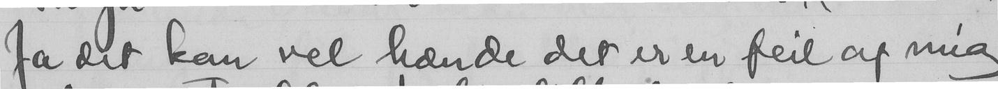Ja det kan vel hænde det er en feil af mig
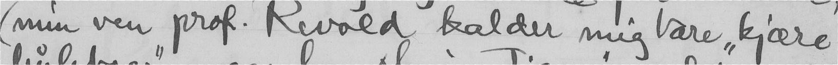(min ven prof. Revold kalder mig bare "kjære
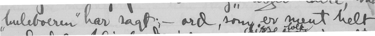"huleboeren" har sagt; - ord, som er ment helt
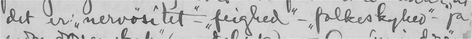det er "nervøsitet" - "feighed" - "folkeskyhed" - ja
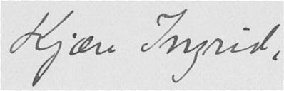Kjære Ingrid,
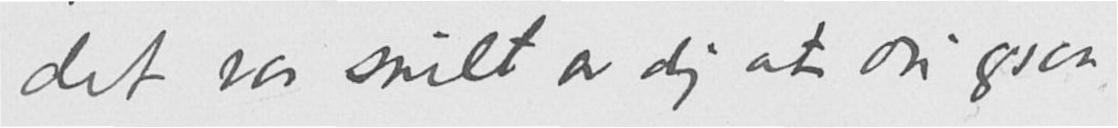det var snilt av dig at du ogsaa
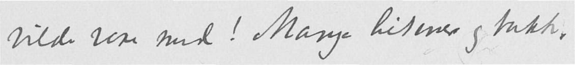vilde være med! Mange hilsener og takk,
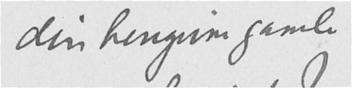din hengivne gamle
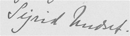Sigrid Undset.
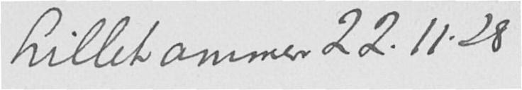Lillehammer 22.11.28
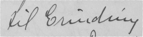til Erindring
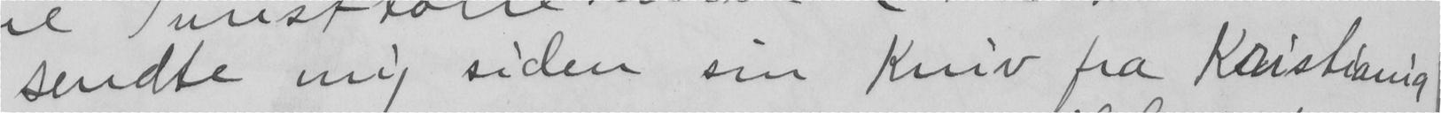sendte mig siden sin Kniv fra Kristiania
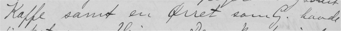Kaffe samt en Ørret som G. havde
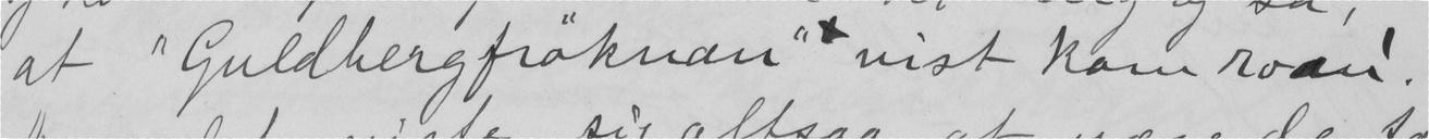at "Guldbergfrøknan" vist kom roan.
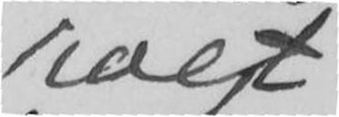roet
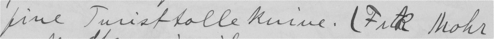fine Turisttolleknive. (Frk Mohr
